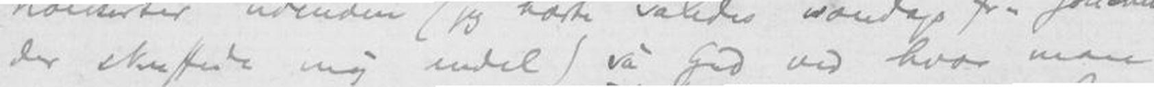der skuffede mig endel) så Gud ved hvor man
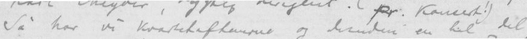Så har vi kvartetaftenerne og desuden en til del
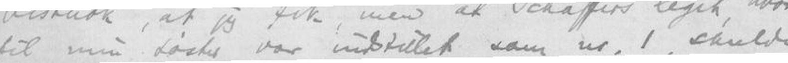til min søster var indstillet som nr. 1, skulde
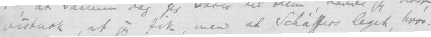vistnok, at jeg fik, men at Schäffers legat, hvor
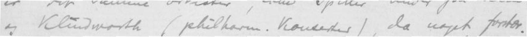og Klindworth (philharm. Konserter), da noget forstær-
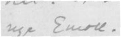nye Emoll.
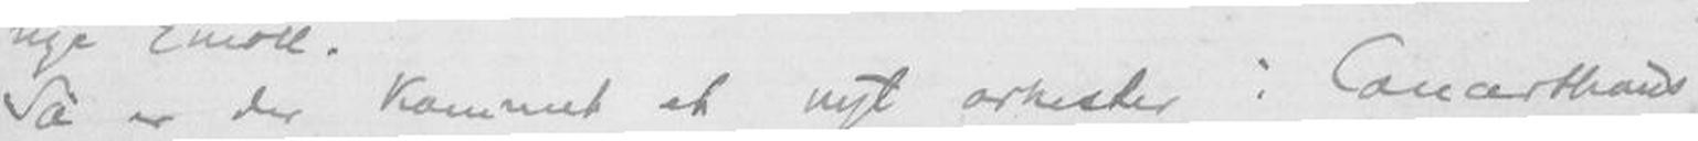Så er der kommet et nyt orkester i Concerthaus
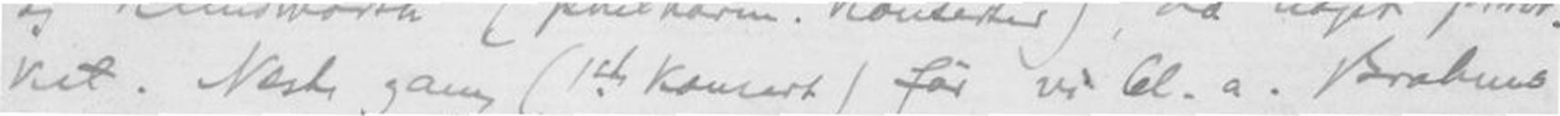ket. Neste gang (14 konsert) før vil bl.a. Brahms
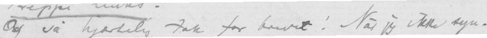Og så hjærtelig tak for brevet! Når jeg ikke syn-
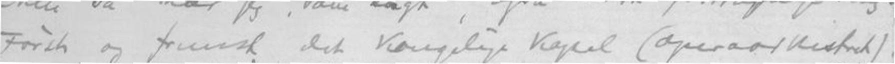Først og fremst det Kongelige Kapel (operaorkesteret).
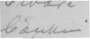bænken".
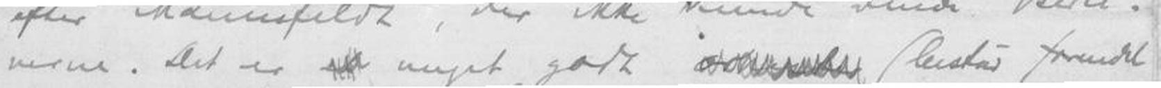nerne. Det er xx meget godt xxxxx (beståd forandet
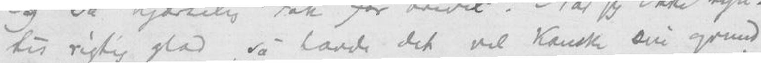tes rigtig glad, så havde det vel kanske sin grund
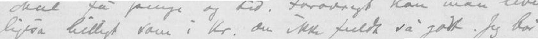ligeså billigt som i Kr. om ikke fuldt så godt. Jeg bor
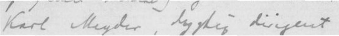Karl Meyder, dygtig dirigent. (I abounement 33 Pf. pr konsert!)
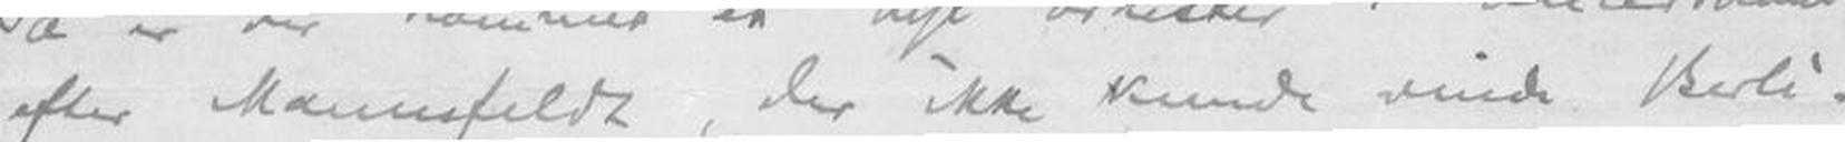efter Mannsfeldt, der ikke kunde vinde Berli-
